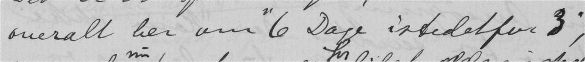overalt ber om "6 Dage istedetfor 3",
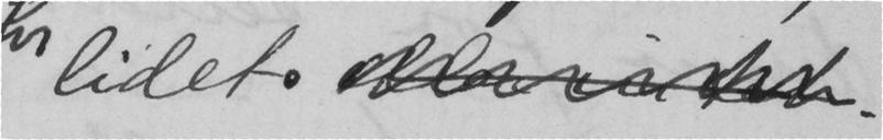lidet.
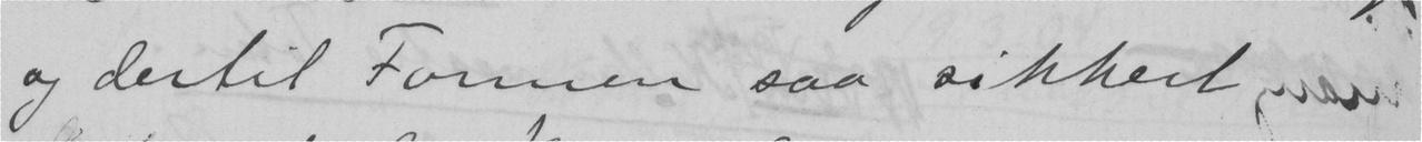og dertil Formen saa sikkert
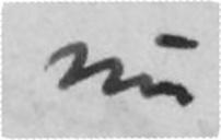nu
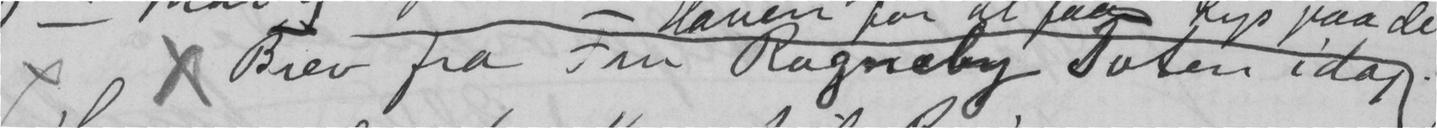x x Brev fra Fru Ragneby Toten idag.
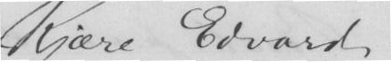Kjære Edvard!
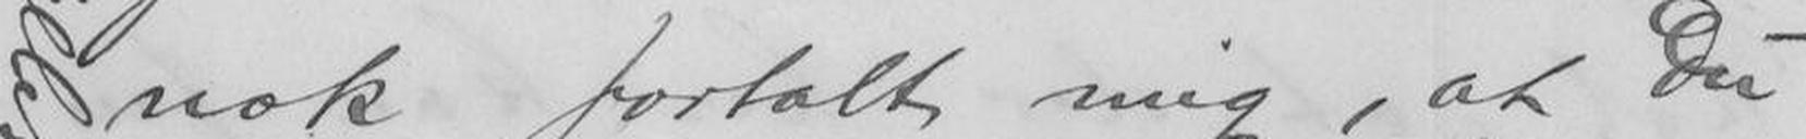nok fortalt mig, at Du
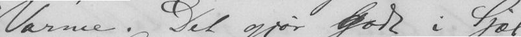Varme. Det gjør godt i Sjæl
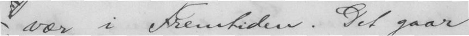vær i Fremtiden. Det gaar,
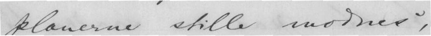planerne stille modnes,
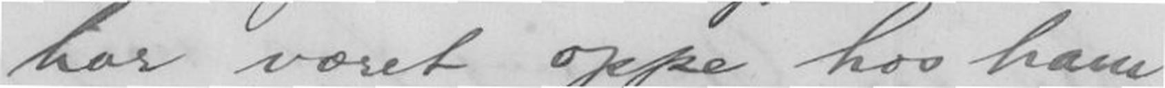har været oppe hos ham
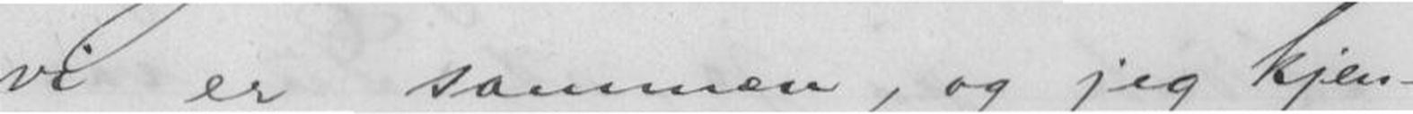vi er sammen, og jeg kjen-
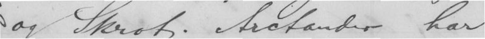og Skrot. Arctander har
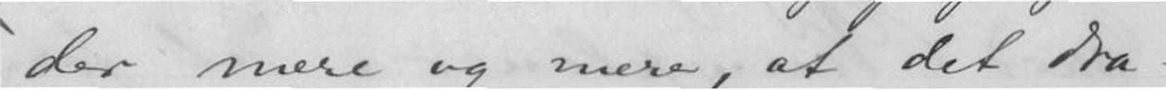der mere og mere, at det dra-
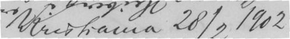Kristiania 28/2 1902.
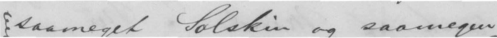saameget Solskin og saamegen
